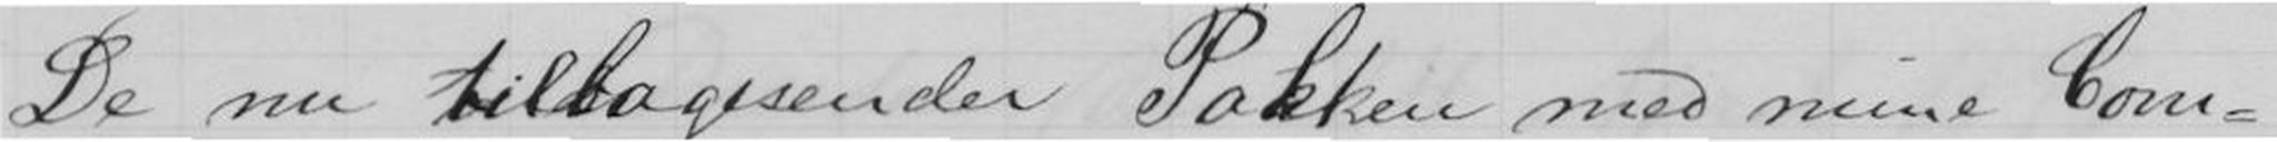De nu tilbagesender Pakke med mine Com-
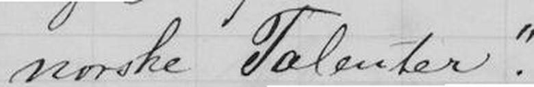norske Talenter".
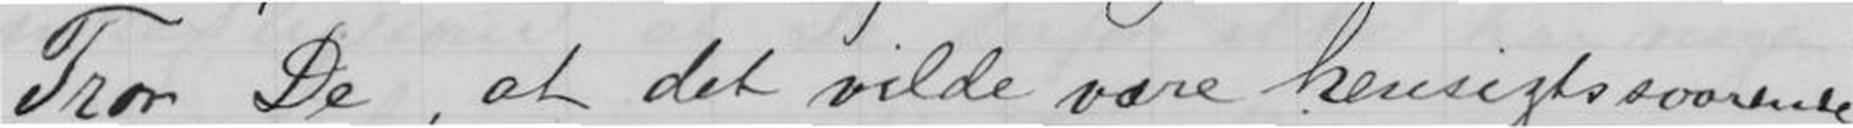Tror De, at det vilde være hensigtssvarende
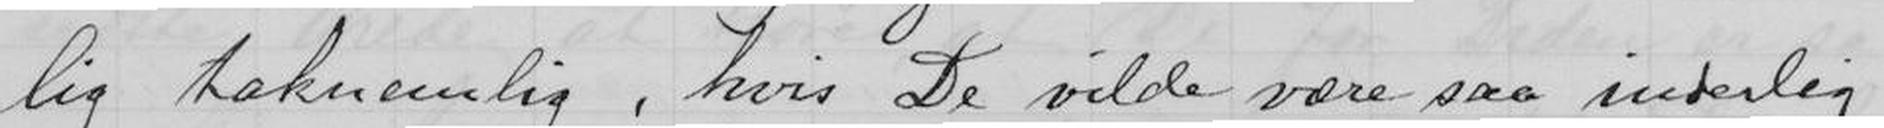lig taknemlig, hvis De vilde være saa inderlig
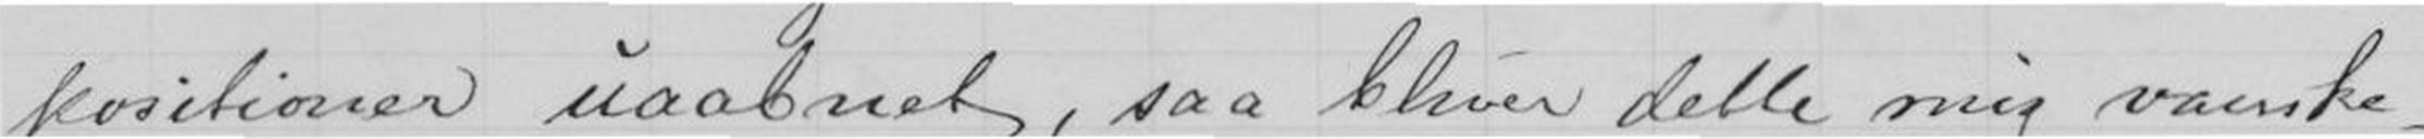positioner uaabnet, saa bliver dette mig vanske-
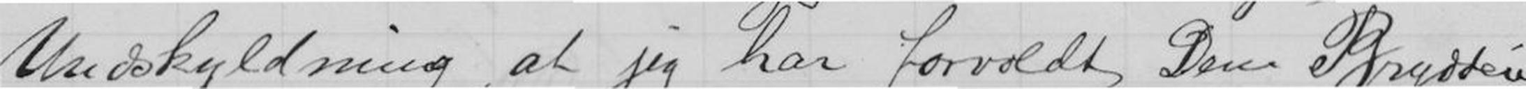Undskyldning at jeg har forvoldt Dem Brydderi
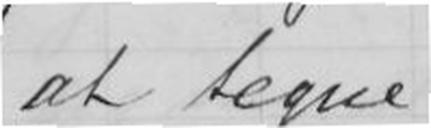at tegne
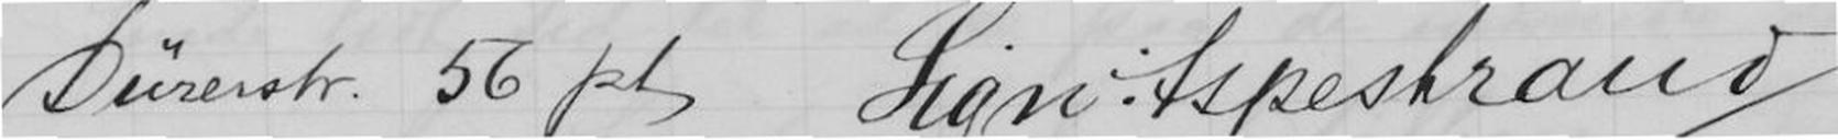Dürerstr. 56 pt.Sigw. Aspestrand
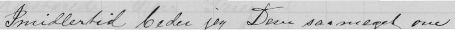Imidlertid beder jeg Dem saameget om
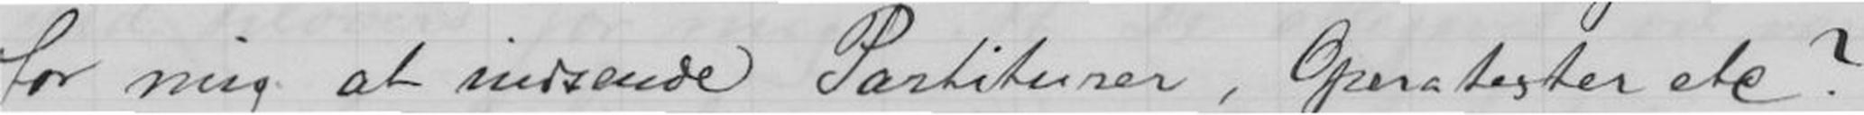for mig at indsende Partiturer, Operatexter etc?
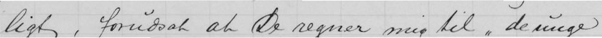ligt, forudsat at De regner mig til "de unge
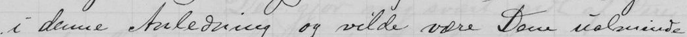i denne Anledning og vilde være Dem ualminde-
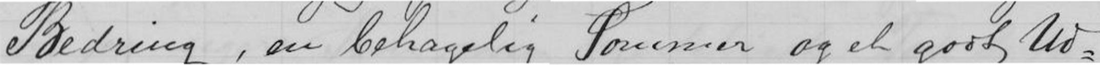Bedring, en behagelig Sommer og et godt Ud-
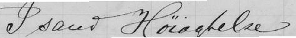I sand Høiagtelse
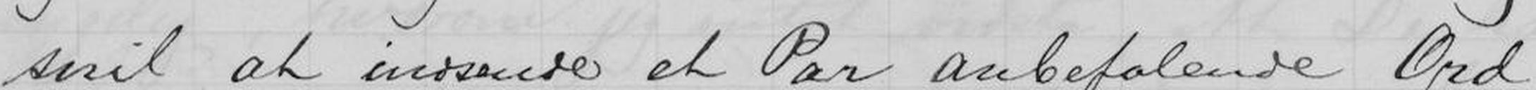snil at indsænde et Par Anbefalende Ord
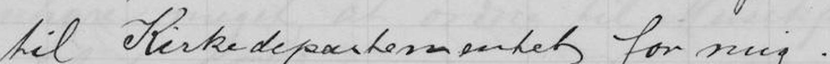til Kirkedepartementet for mig.
